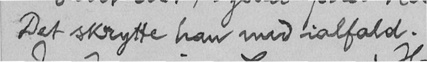Det skrytte han med ialfald.
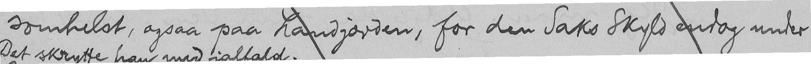somhelst, ogsaa paa Landjorden, for den Saks Skyld endog under
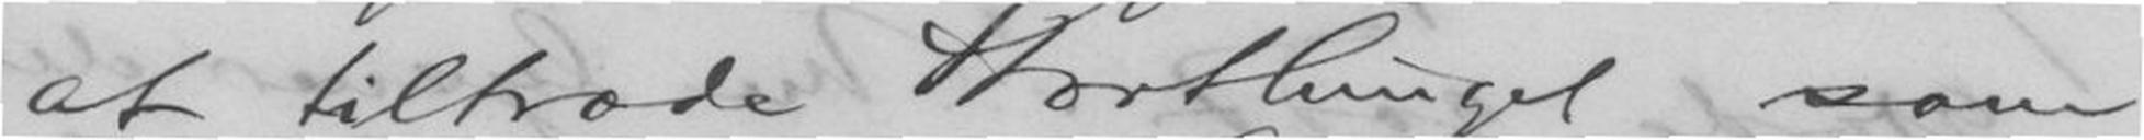at tiltræde Storthinget som
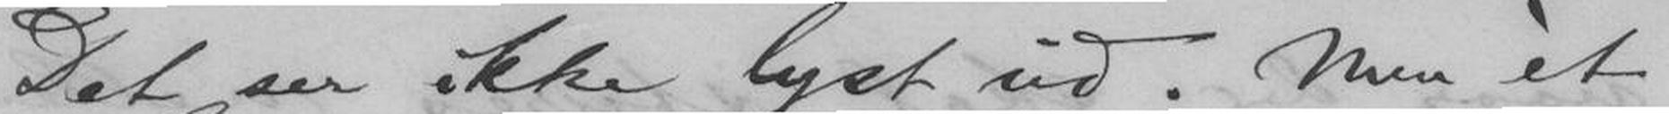Det ser ikke lyst ud. Men èt
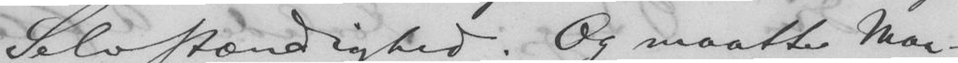Selvstændighed. Og maatte Maa-
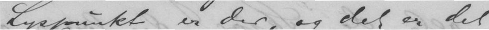Lyspunkt er der, og det er det
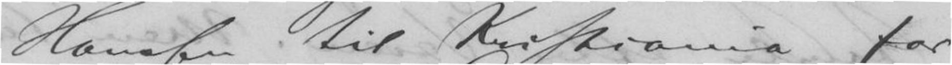Hanssen til Kristiania for
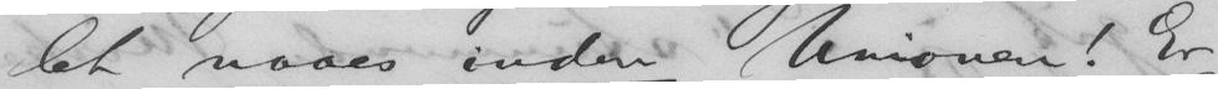let naaes inden Unionen! Er
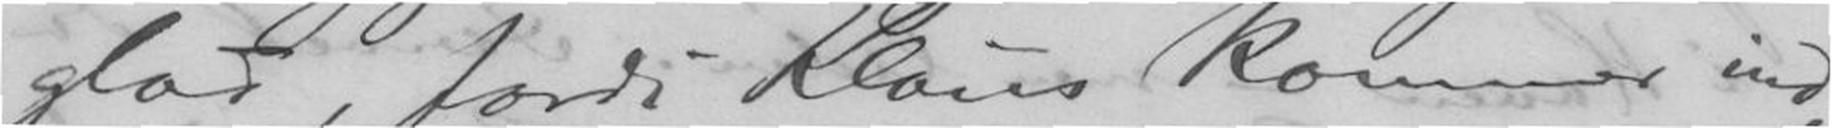glad, fordi Klaus kommer ind,
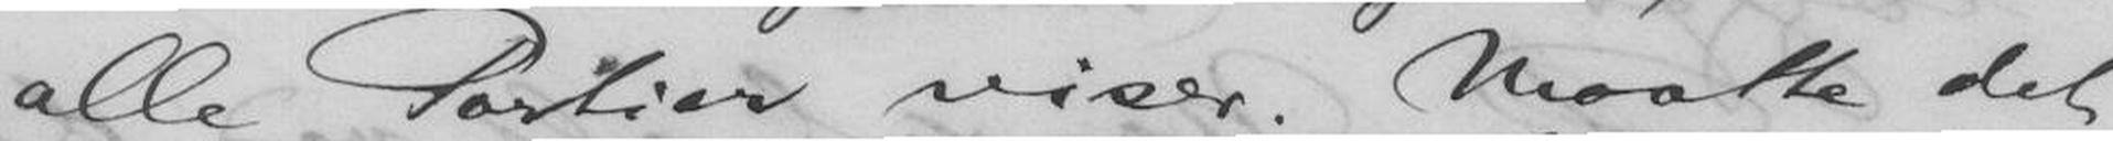alle Partier viser. Maatte det
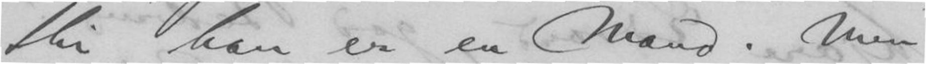thi han er en Mand. Men
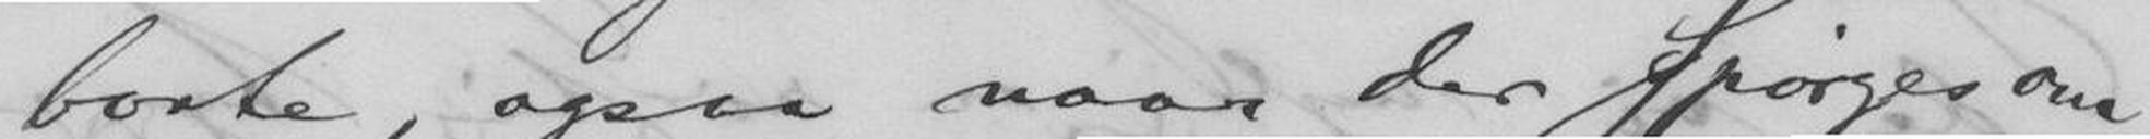borte, ogsaa naar der Spørges om
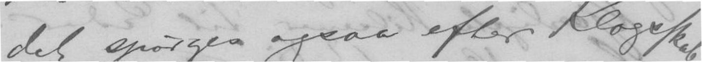det spørges ogsaa efter Klogskab,
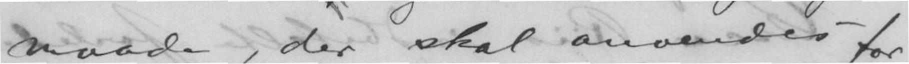maade, der skal anvendes for
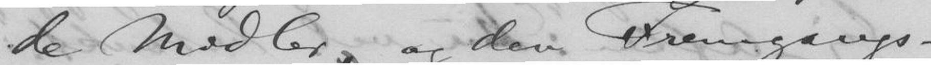de Midler, og den Fremgangs-
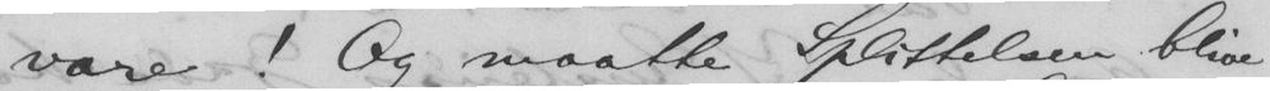vare! Og maatte Splittelsen blive
